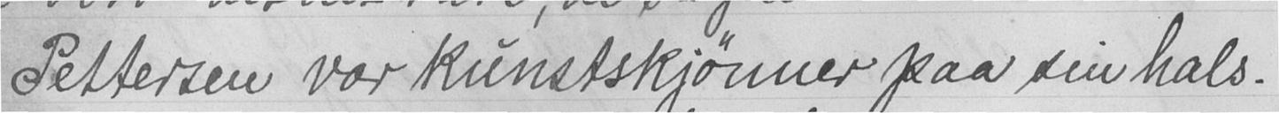Pettersen var kunstskjønner paa sin hals.
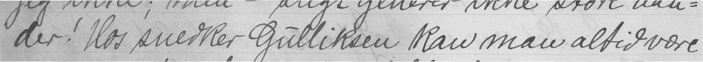der! Hos snedker Gulliksen kan man altid være
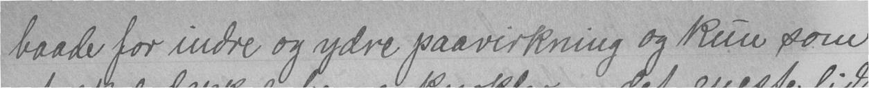baade for indre og ydre paavirkning og kun som
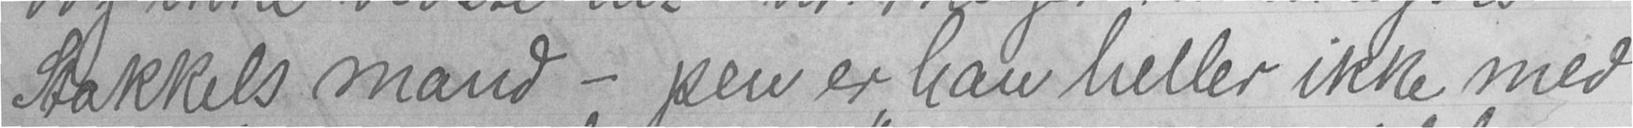Sakkels mand - pen er han heller ikke med
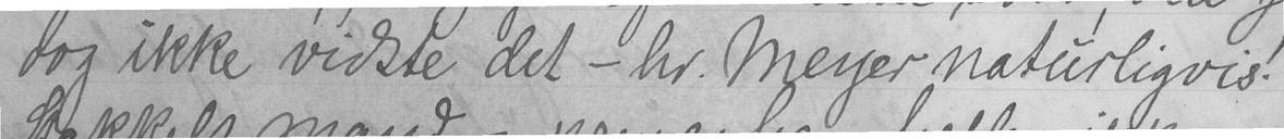dog ikke vidste det - hr. Meyer naturligvis!
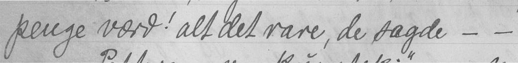penge værd! alt det rare, de sagde - -
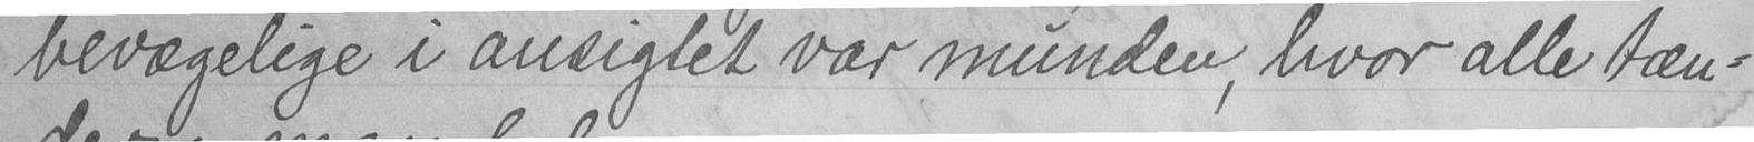bevægelige i ansiglet var munden, hvor alle tæn-
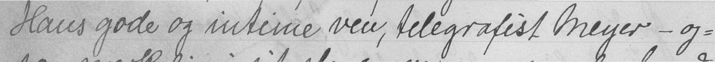Hans gode og intime ven, telegrafist Meyer - og-
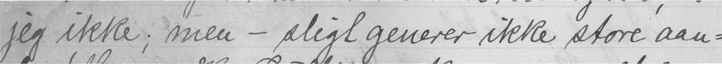jeg ikke; men - sligt generer ikke store aan-
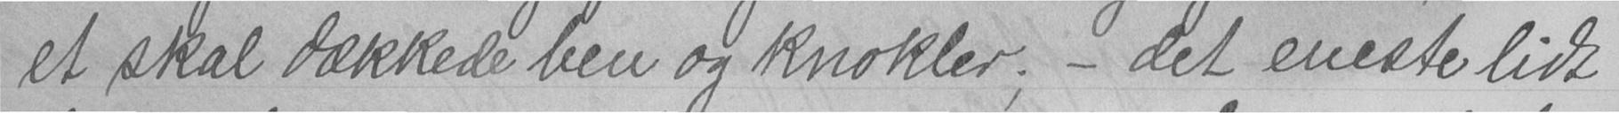et skal dækkede ven og knokler; - det eneste lidt
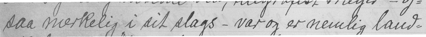saa merkelig i sit slags - var og er nemlig land-
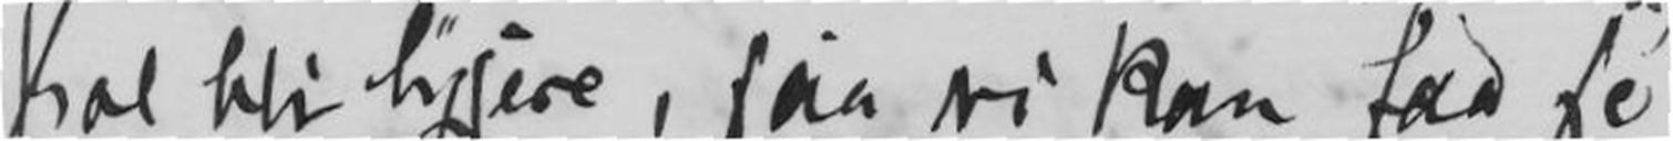skal bli lysere, saa vi kan faa se
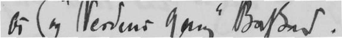os og "Verdens gang" Besked.
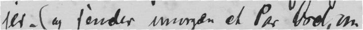jer - og sender imorgen et Par Ord, om
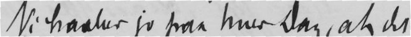Vi haaber jo paa hver Dag, at det
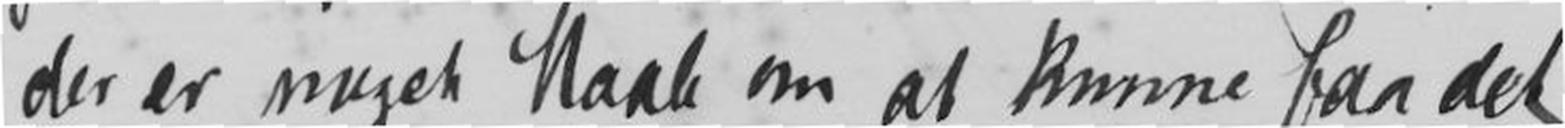der er noget Haab om at kunne faa det
